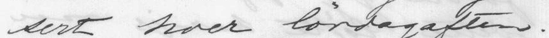sert hver lørdagaften.
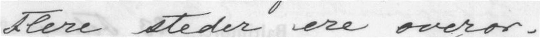Flere steder ere overor-
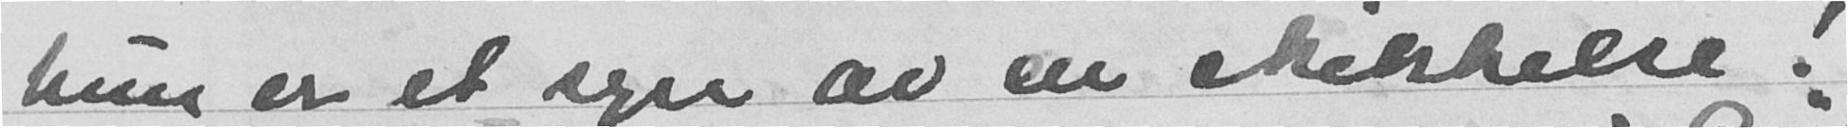hun er et syn av en skikkelse!
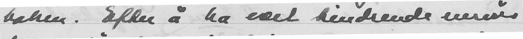boken. Efter å ha vært tindrende nervøs
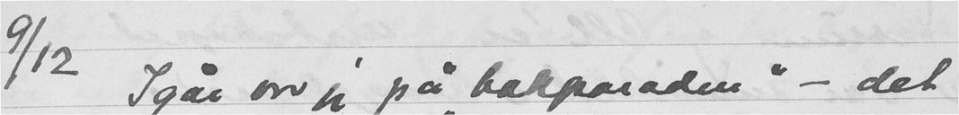9/12 	Igår var jeg på "bokparaden" - det
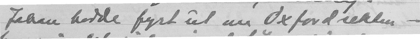Johan hadde fyrt ut om Oxfordsekten -
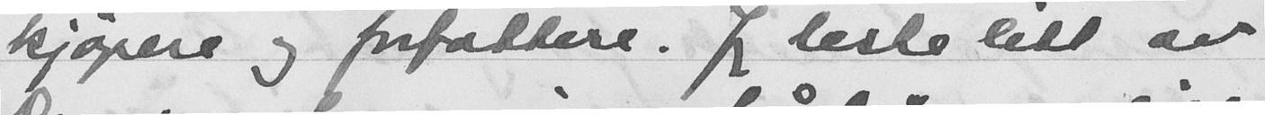kjøpere og forfattere. Jeg leste litt av
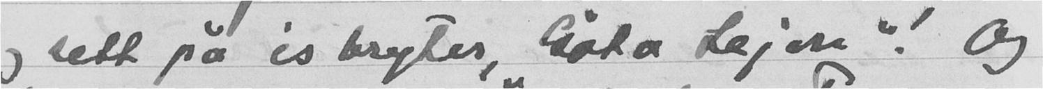og sett på isbryter, "Gåta Leire". Og
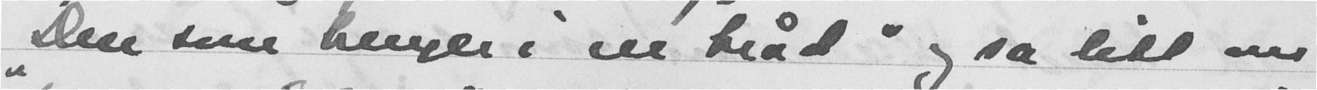"Den som henger i en tråd" og så litt om
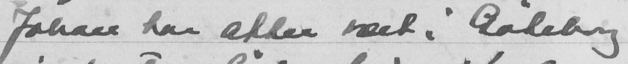Johan har atter vært i Gøteborg
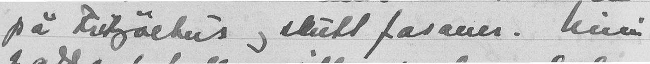på Fretzolhus og skutt fasaner. Men
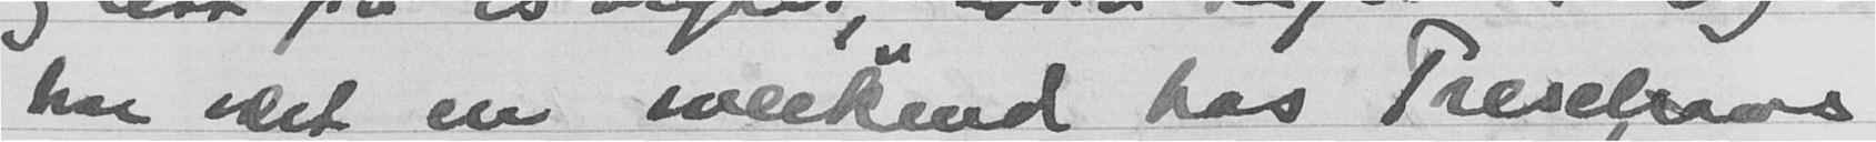har vært en weekend hos Treschows
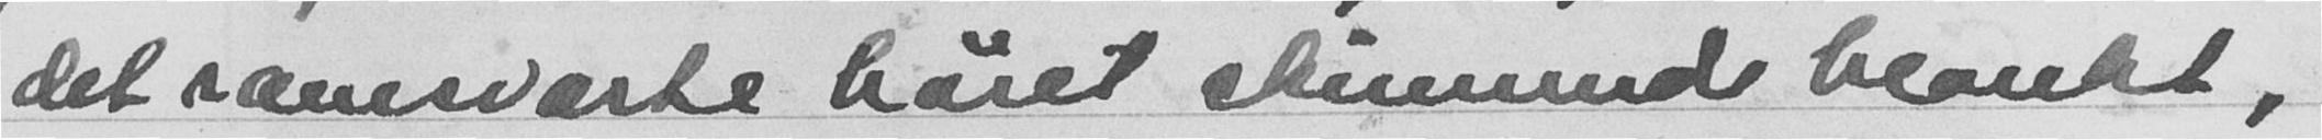det ravnsvarte håret skinnende blankt,
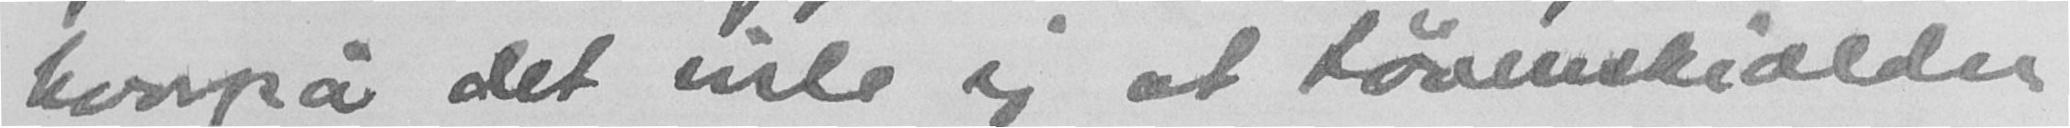hvorpå det viste sig at tøvenskiælder
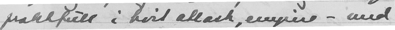praktfull i hvit stlæsk, emyene - med
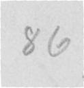86
